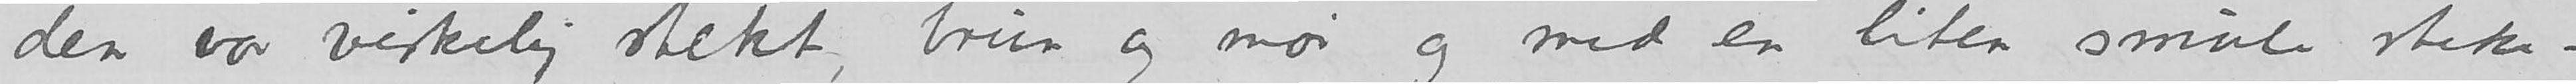den var virkelig stekt, brun og mør og med en liten smule steke-
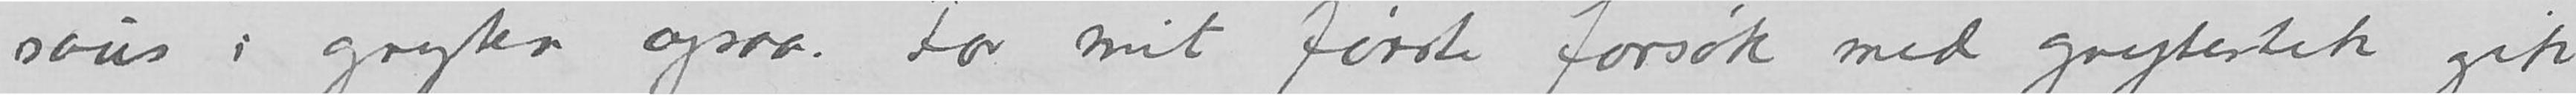saus i gryten ogsaa. For mit første forsøk med grystestek gik
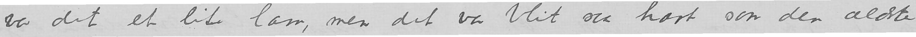var det et lite lam, men det var blit saa hart som den ældste
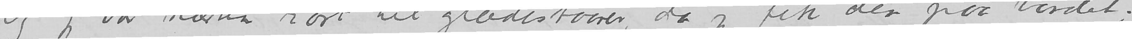Og jeg var næsten rørt til glædestaarer, da jeg fik den paa bordet;
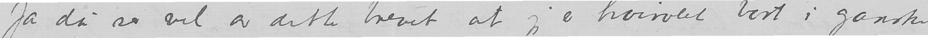Ja du ser vel av dette brevet at jeg er hvirvlet bort i ganske
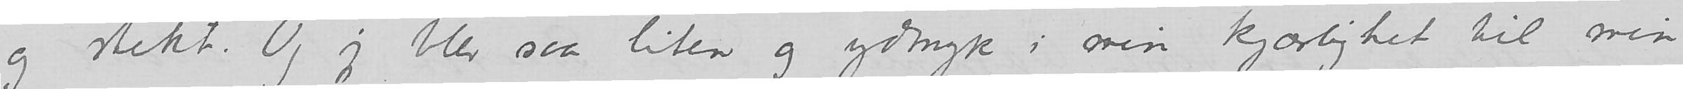og stekt. Og jeg blev saa liten og ydmyk i min kjærlighet til min
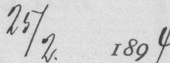25/2 189
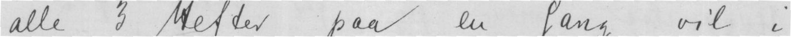alle 3 Hefter paa en gang vil i
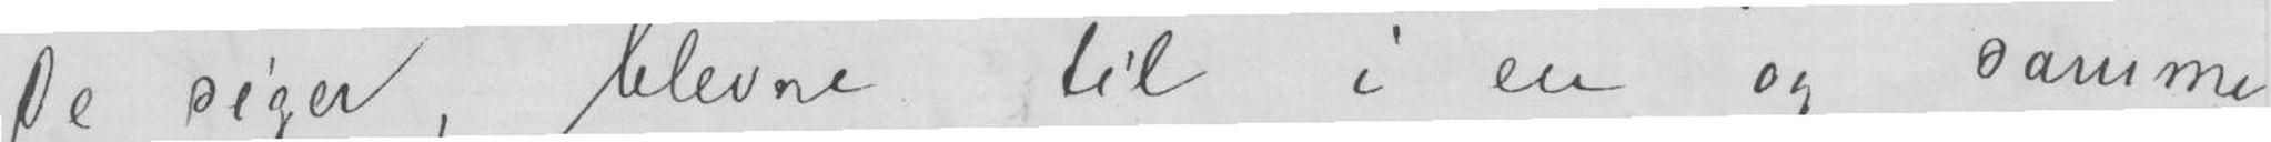De siger, blevne til i en og samme
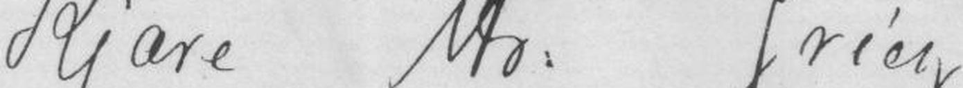Kjære Hr. Grieg!
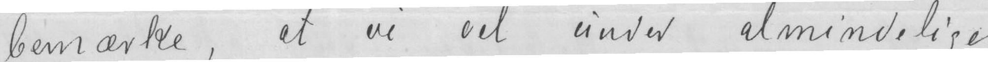bemærke, at vi vel under almindelige
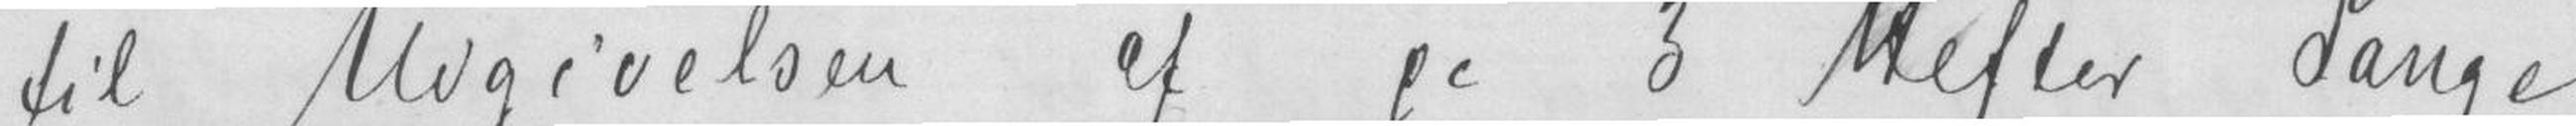til Udgivelsen af de 3 Hefter Sange
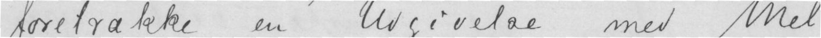foretrække en Udgivelse med Mel-
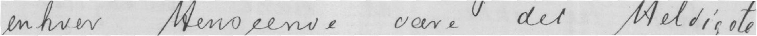enhver Henseende være det Heldigste.
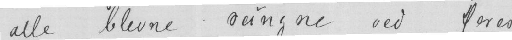alle blevne sungne ved Deres
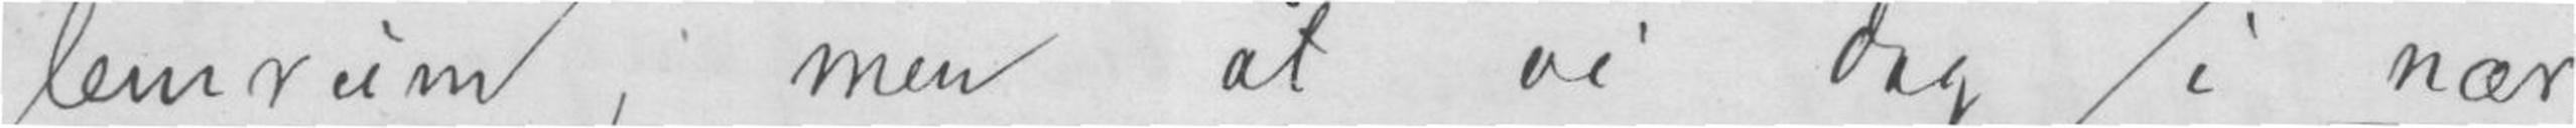lemrum, men at vi dog i nær-
